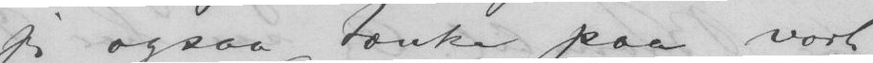jeg ogsaa tænke paa vort
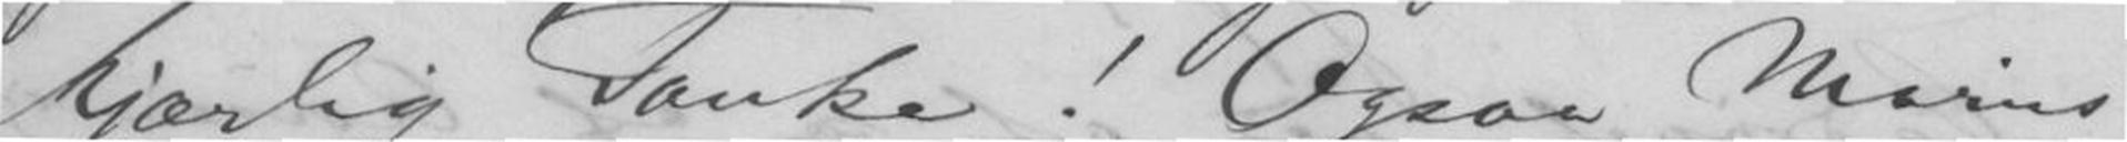kjærlig Tanke! Ogsaa Maries
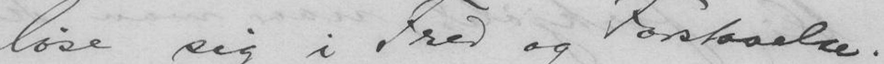løse sig i Fred og Forstaaelse.
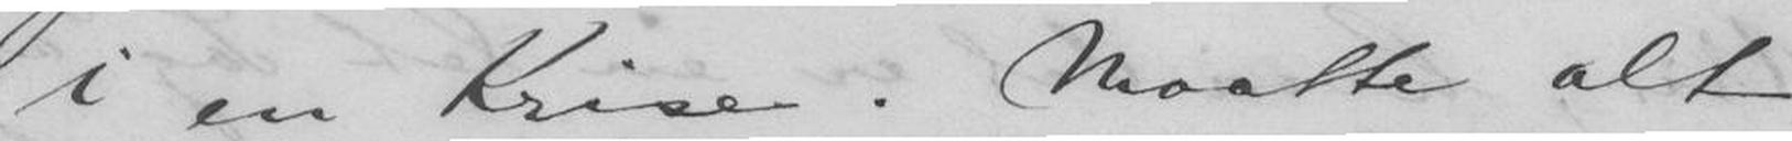i en Krise. Maatte alt
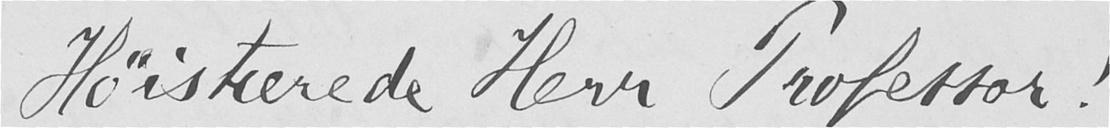Høistærede Herr Professor!
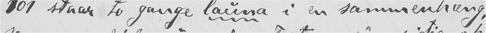101 staar to gange launa i en sammenhæng,
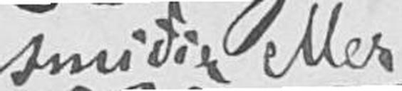smiðir eller
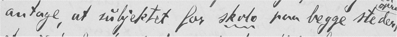antage, at subjektet for skolo paa begge steder
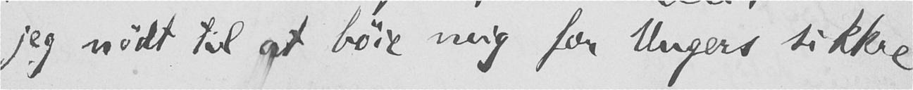jeg nødt til at bøie mig for Ungers sikkre
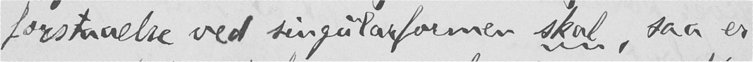forstaaelse ved singularformen skal, saa er
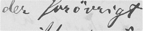der forøvrigt
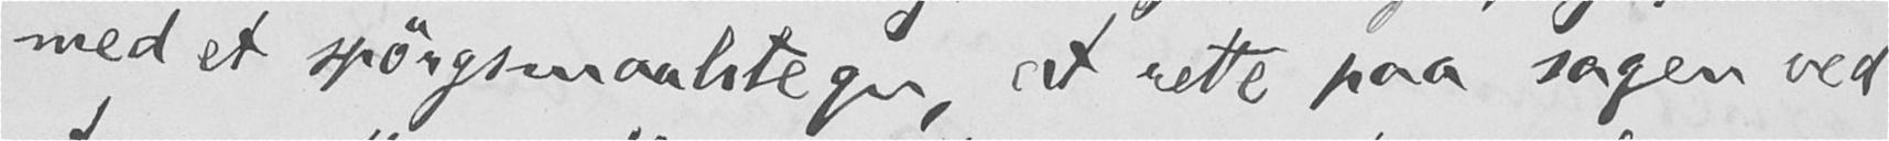med et spørgsmaalstegn, at rette paa sagen ved
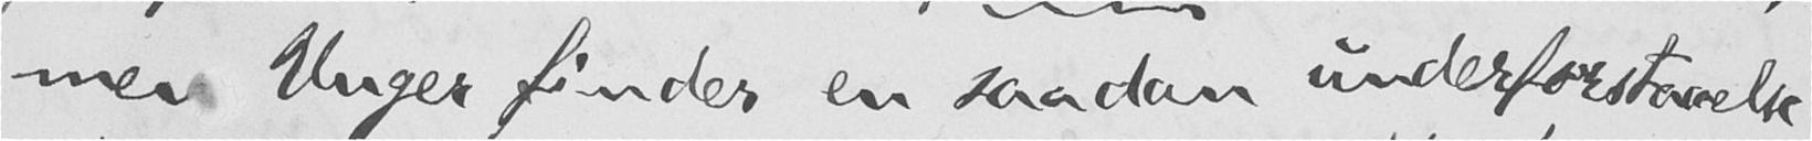men Unger finder en saadan underforstaaelse
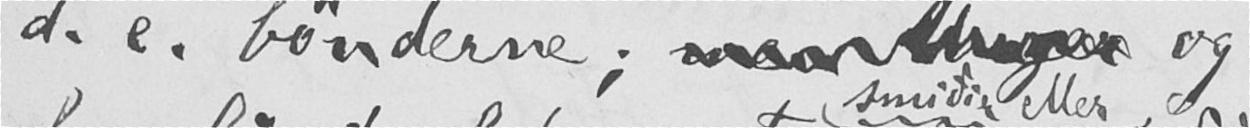d. e. bønderne;  og
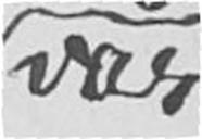var
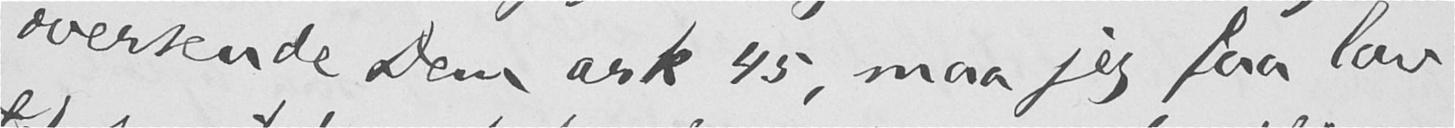oversende Dem ark 45, maa jeg faa lov
